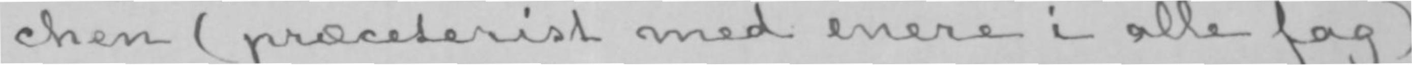chen (præceterist med enere i alle fag)
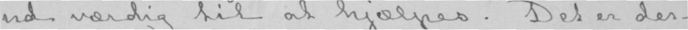ud værdig til at hjælpes. Det er der-
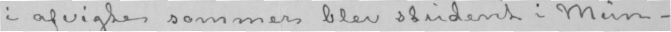i afvigte sommer blev student i Mün-
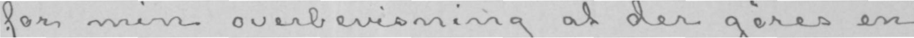for min overbevisning at der gøres en
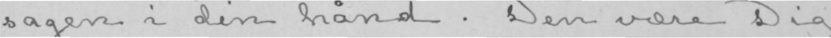sagen i din hånd. Den være Dig
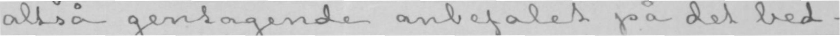altså gentagende anbefalet på det bed-
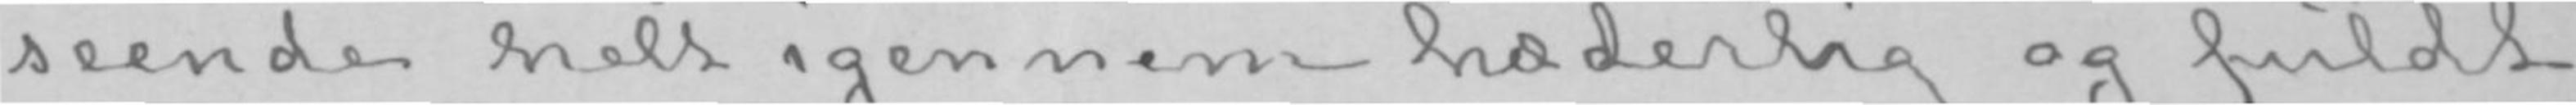seende helt igennem hæderlig og fuldt
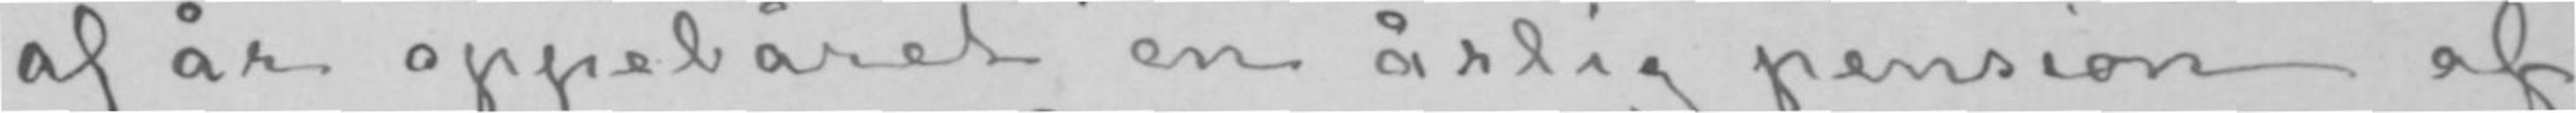af år oppebåret en årlig pension af
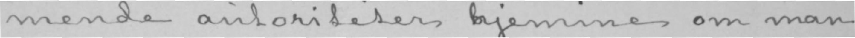mende autoriteter ohjemme om man
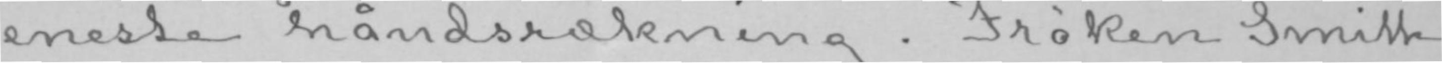eneste håndsrækning. Frøken Smith
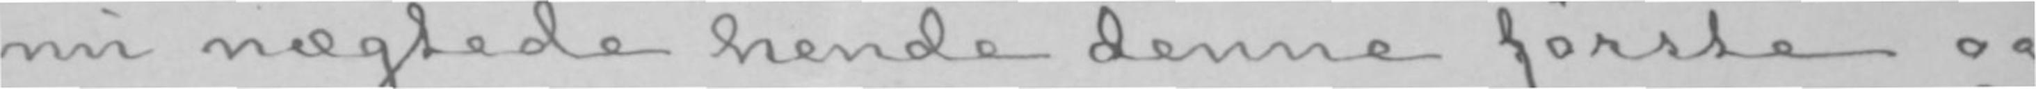nu nægtede hende denne første og
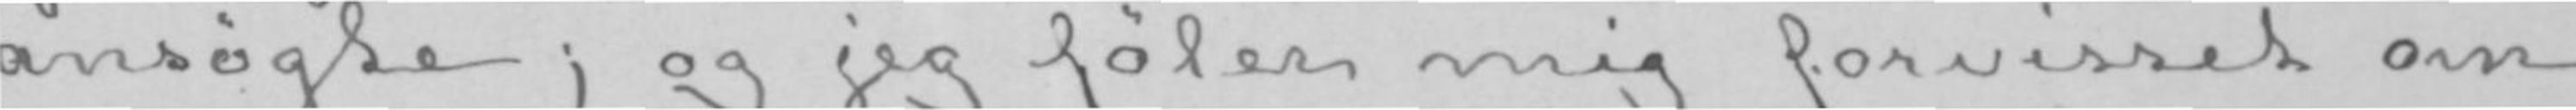ansøgte; og jeg føler mig forvisset om
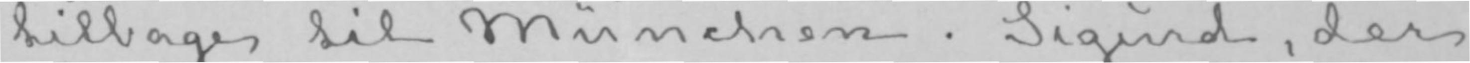tilbage til München. Sigurd, der
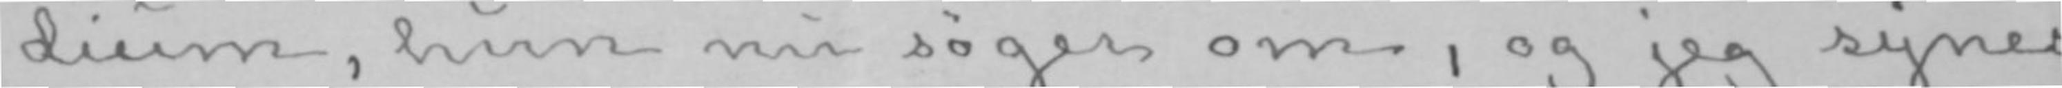dium, hun nu søger om, og jeg synes
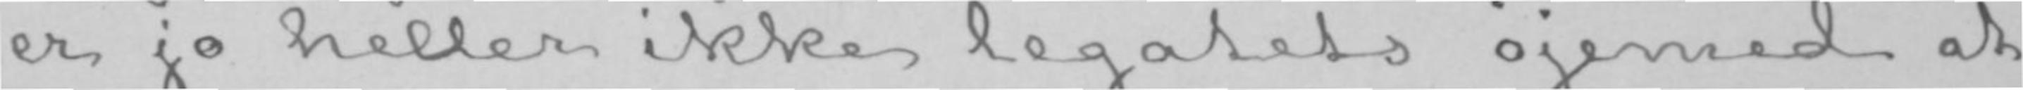er jo heller ikke legatets øjemed at
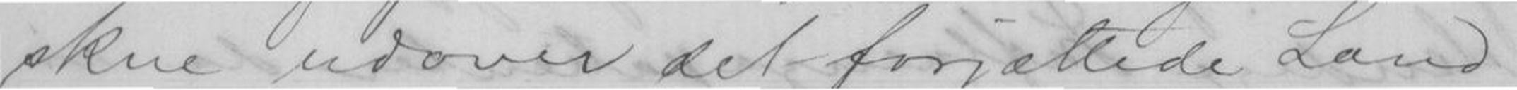skue udover det forjættede Land
